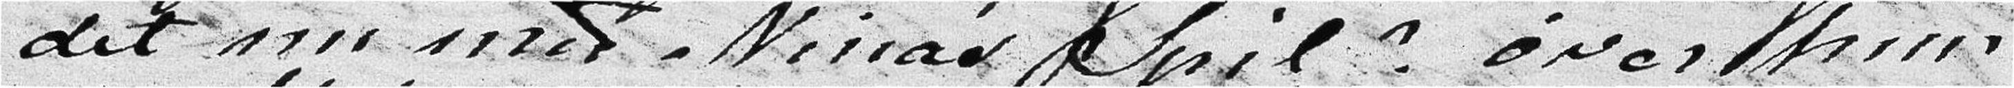det nu med Ninas Spil? øver hun
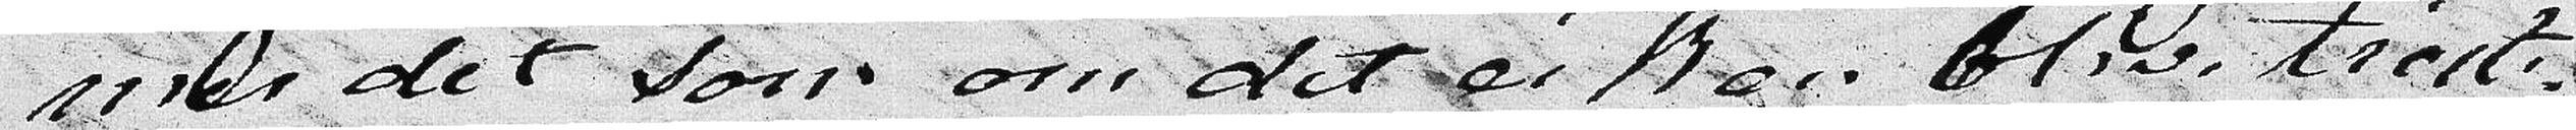mer det som om det ei kan blive trøste-
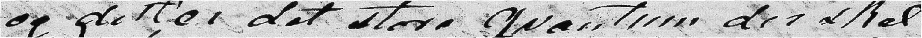og det er det store Qvantum der skal
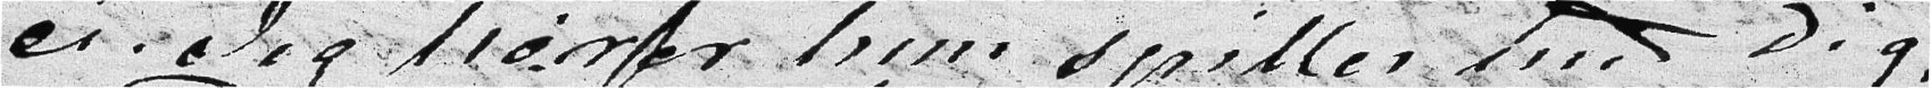ei. Jeg hører hun spiller med Dig,
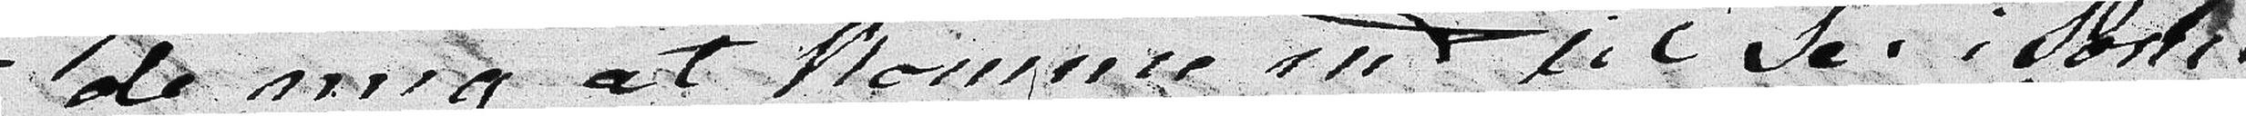de mig at komme ned til Jer i Som-
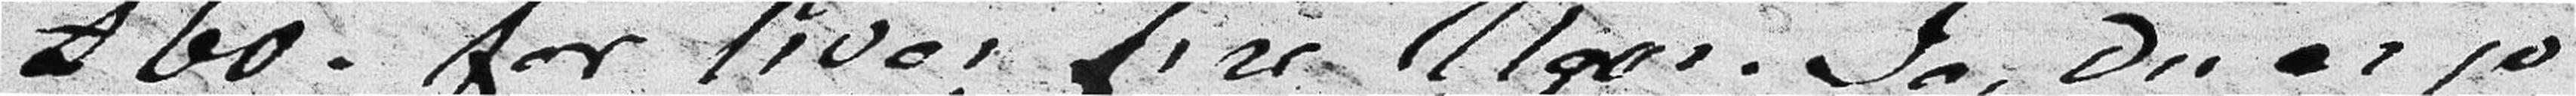à 60 for hver fire Uger. Ja, Du er jo
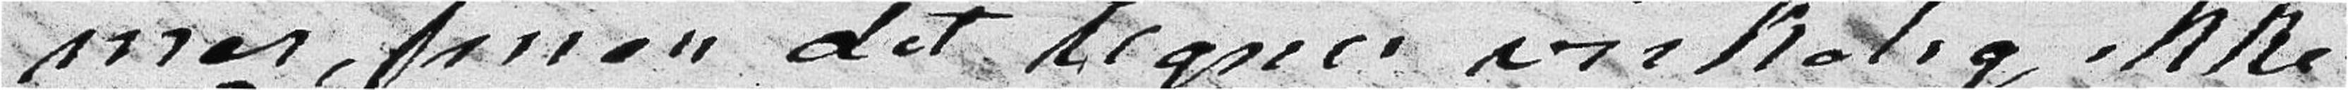mer, men det tegner virkelig ikke
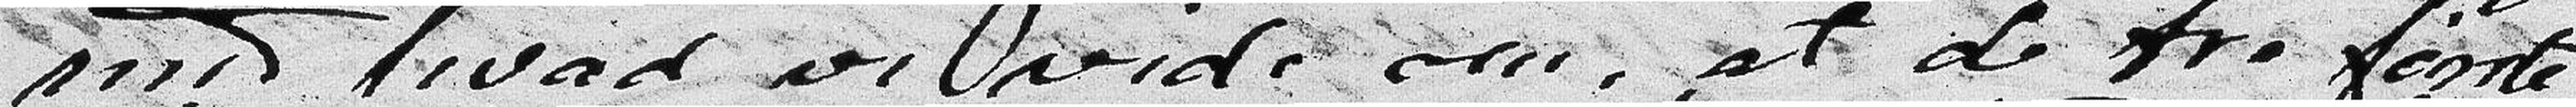med hvad vi vide om, at de tre første
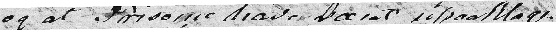og at Priserne have været upaaklage-
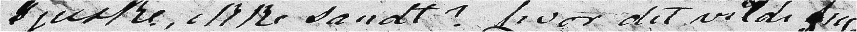sjuske, ikke sandt? hvor det vilde fry-
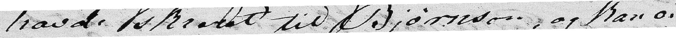havde skrevet til Bjørnson, og kan ei
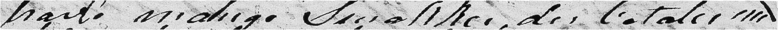have mangen Smakker, der betaler med
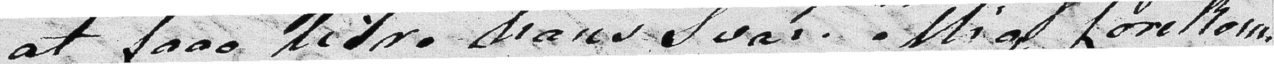at faae høre hans Svar. Mig forekom-
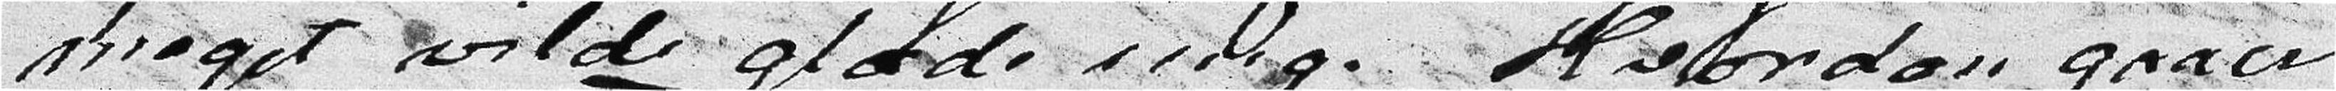meget vilde glæde mig. Hvordan gaaer
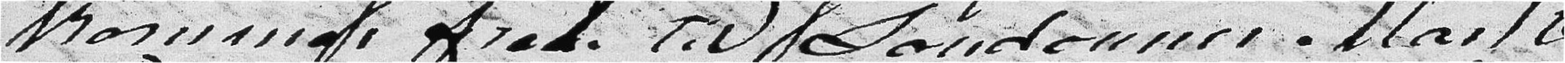kommer frem til Londonner Marke-
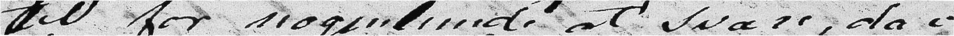til for nogenlunde at svare, da vi
